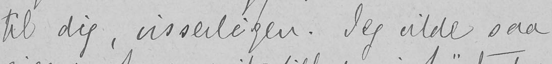til dig, visserligen. Jeg vilde saa
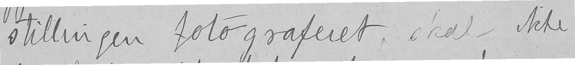stillingen fotograferet, skal ikke
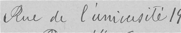Rue de l'université 19
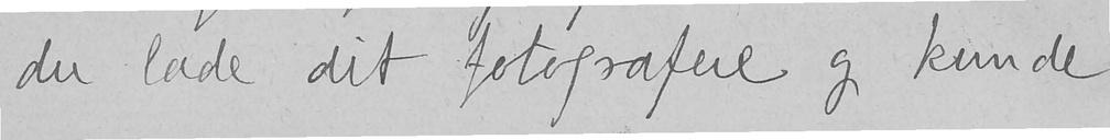du lade dit fotografere og kunde
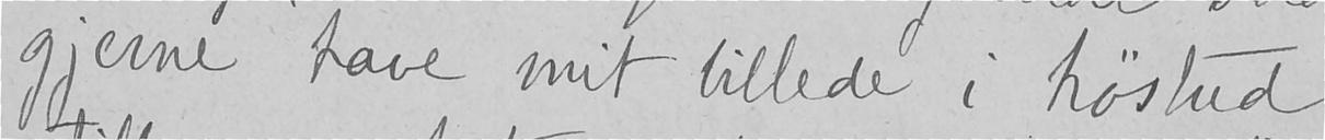gjerne have mit billede i høstud-
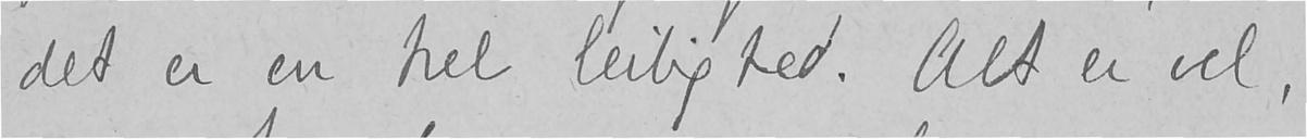det er en hel leilighed. Alt er vel,
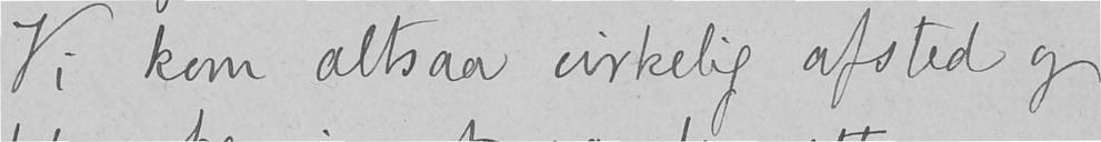Vi kom altsaa virkelig afsted og
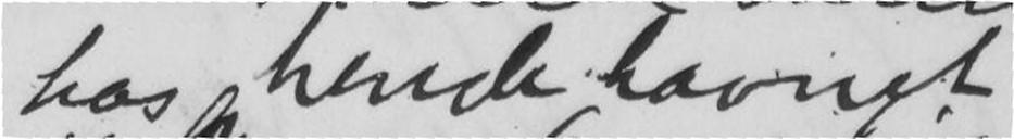hos hende havnet
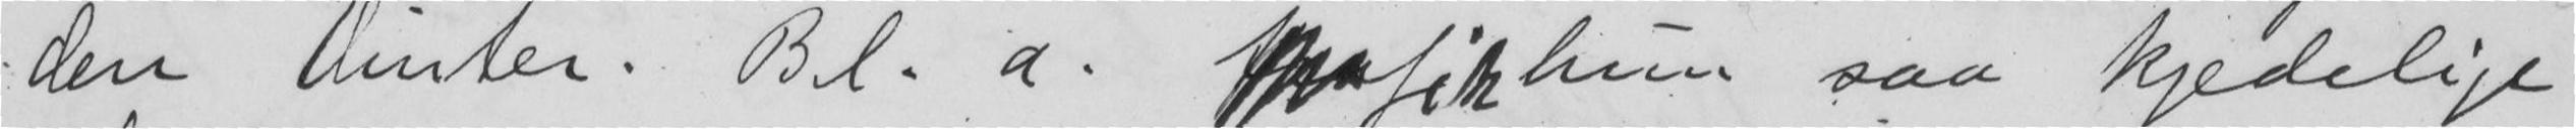den Vinter. Bl. a.  fik hun saa kjedelige
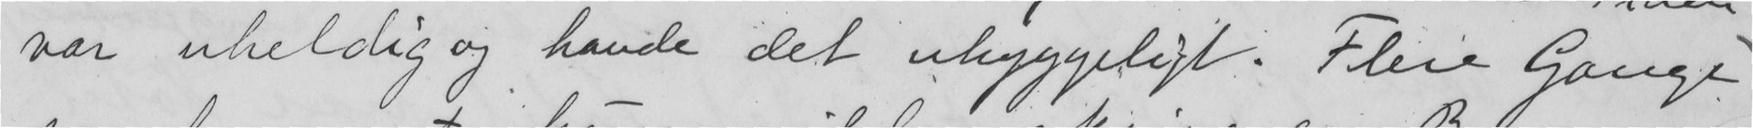var uheldig og havde det uhyggeligt. Flere Gange
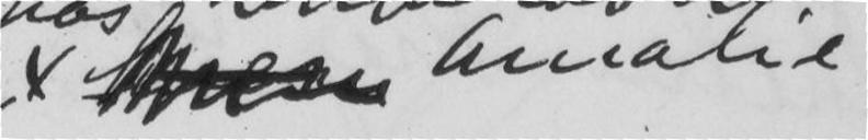Amalie
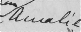Amalie
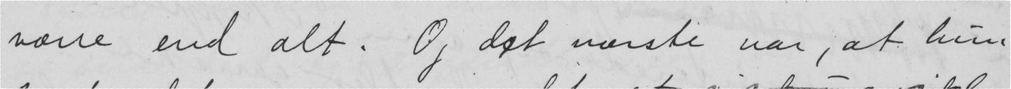værre end alt. Og det værste var, at hun
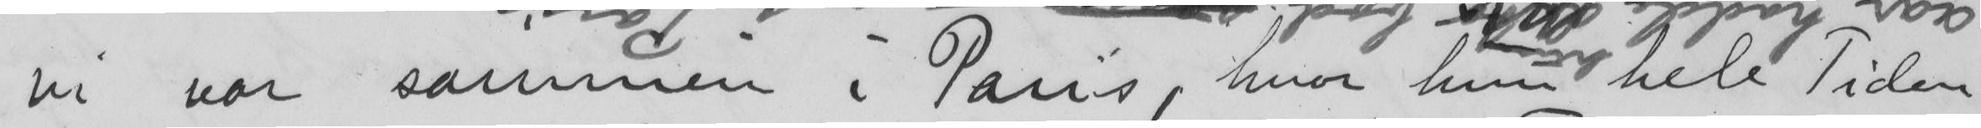vi var sammen i Paris, hvor hun hele Tiden
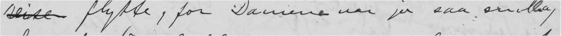flytte, for Danene var jo saa snille og
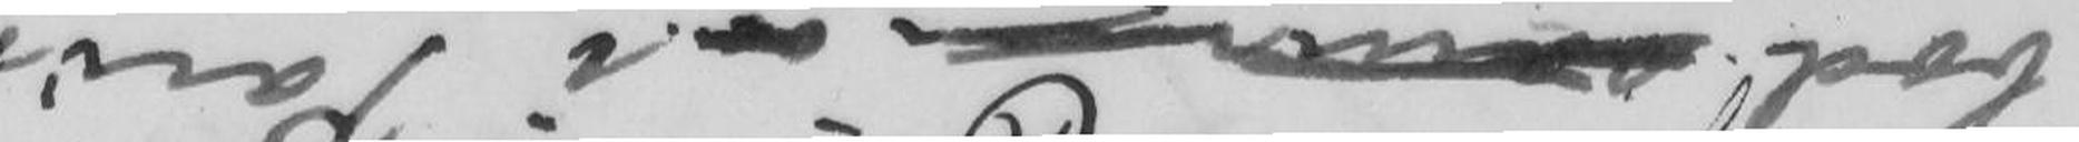bod  i Paris.
